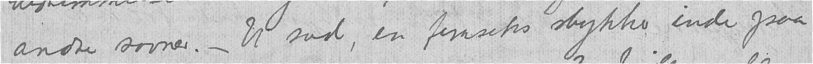andre savner. - Vi sad, en fem-seks stykker inde paa
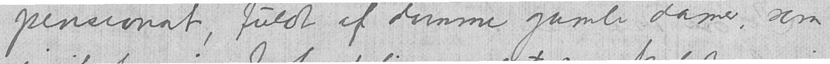pensionat, fuldt af dumme gamle damer, som
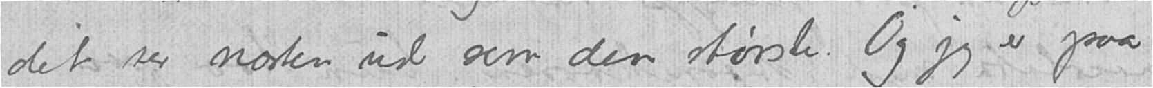det ser nesten ud som den største. Og jeg er paa
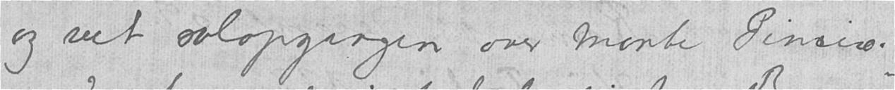og seet soloppgangen over Monte Pincio. -
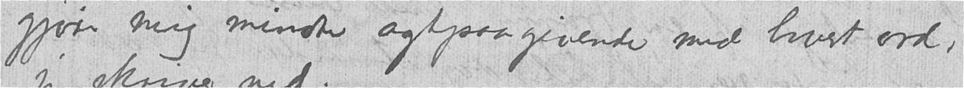gjøre mig mindre agtpaagivende med hvert ord,
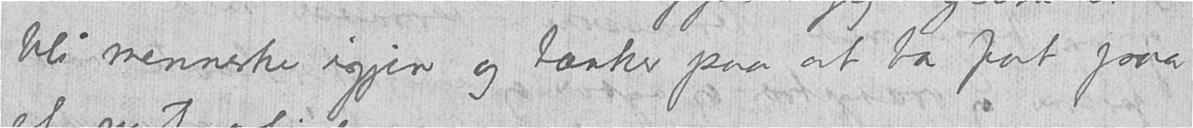bli menneske igjen og tænker paa at ta fat paa
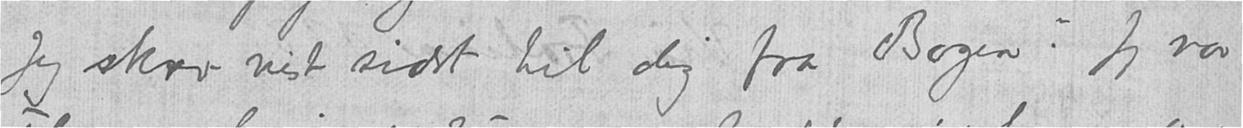Jeg skrev vel sidst til dig fra Bozen? Jeg var
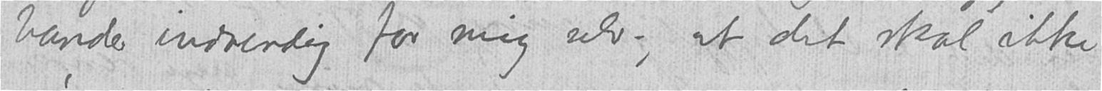bander indvendig for mig selv -, at det skal ikke
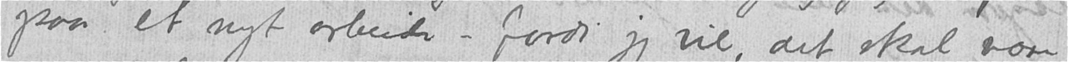paa et nyt arbeide - fordi jeg vil, det skal være
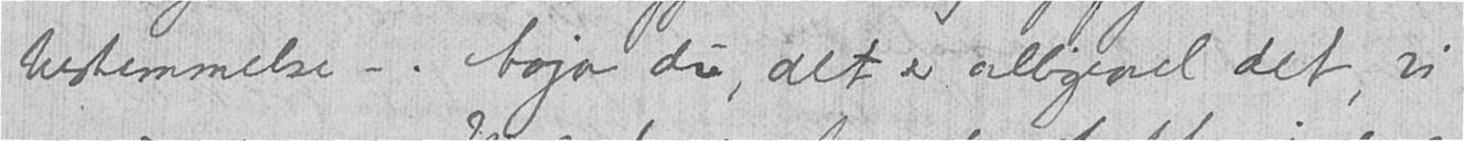bestemmelse -. Aaja du, det er alligevel det, vi
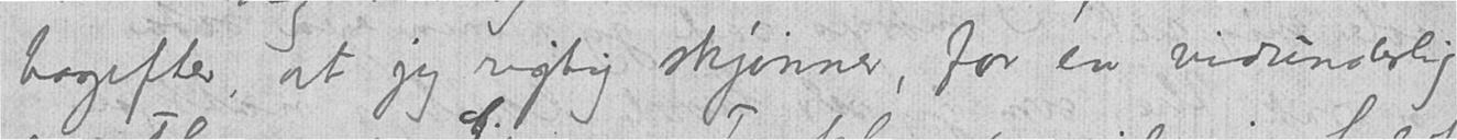bagefter, at jeg rigtig skjønner, for en vidunderlig
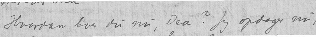Hvordan bor du nu, Dea? Jeg opdager nu,
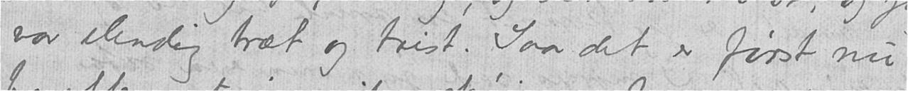var elendig træt og trist. Saa det er først nu
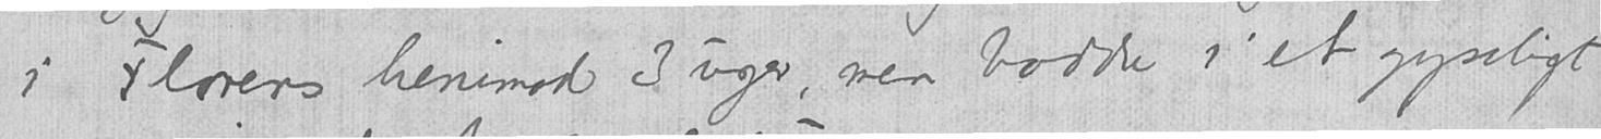i Florens henimod 3 uger, men bodde i et gyseligt
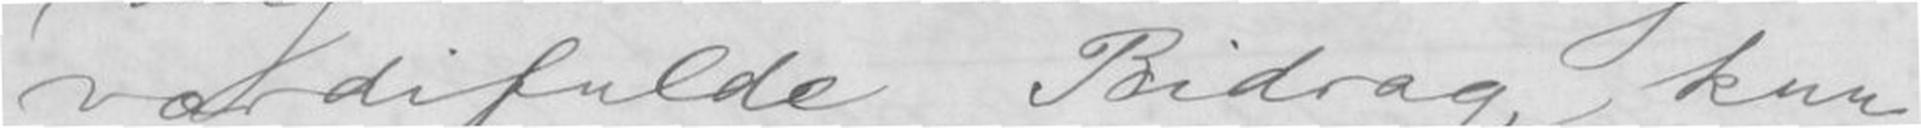værdigfulde Bidrag, kun
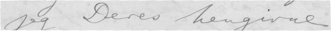jeg Deres hengivne
